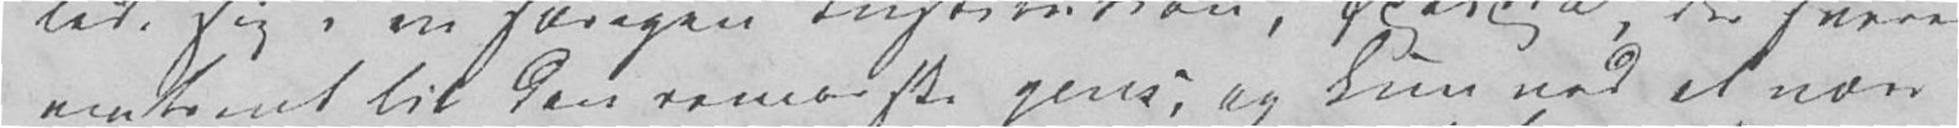omtrent til den romerske gens, og kun ved at være
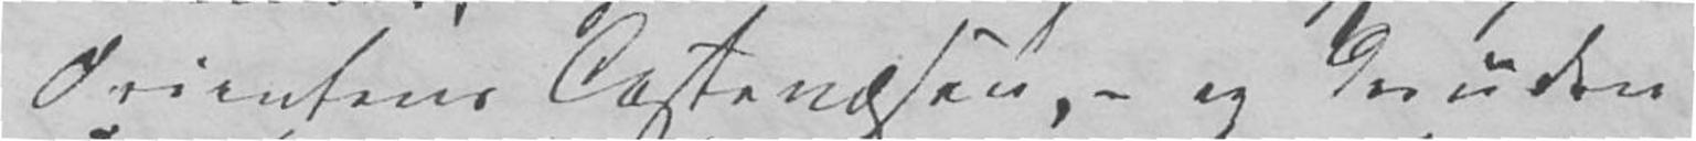Orientens Castevæsen, - og desuden
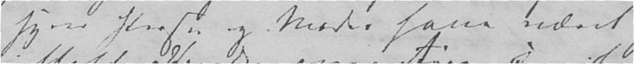X Perser og Moder have været
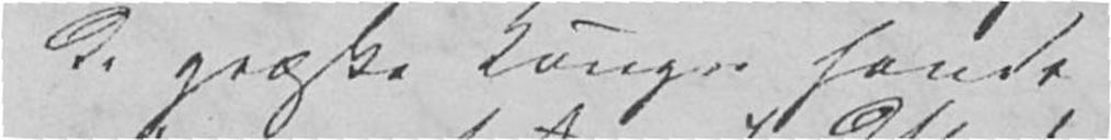de græske Konger havde
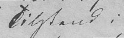Tilstand i
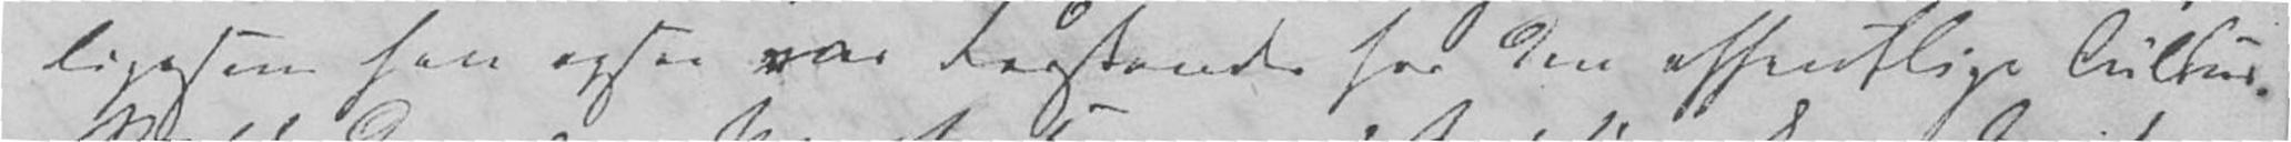ligesom han ogsaa var Forstander for den offentlige Cultur.
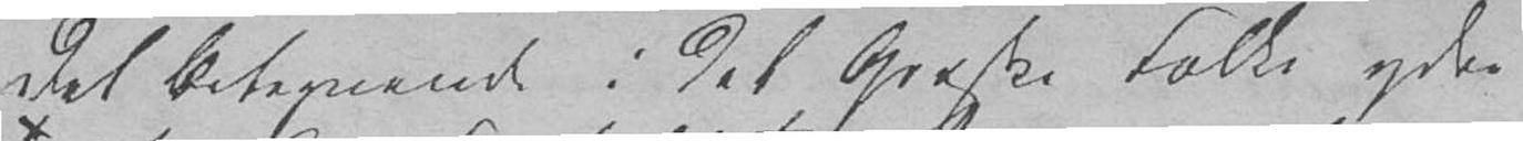Det Betegnende i det Græske Folks ydre
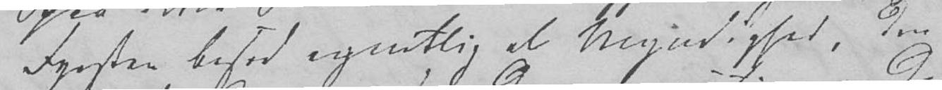Fyrsten besad egentlig al Myndighed, den
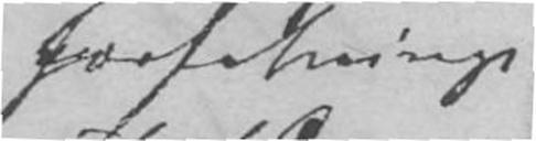forfatnings-
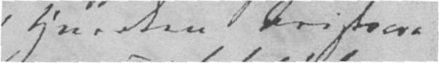Hverken Aristocra-
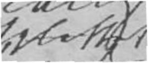slet
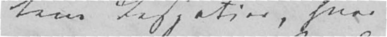tens Despotie, hvor
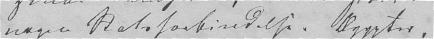nogen Statsforbindelse. Ægtypter,
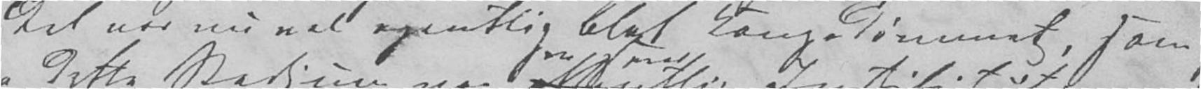Det var nu vel egentlig blot Kongedømmet, som
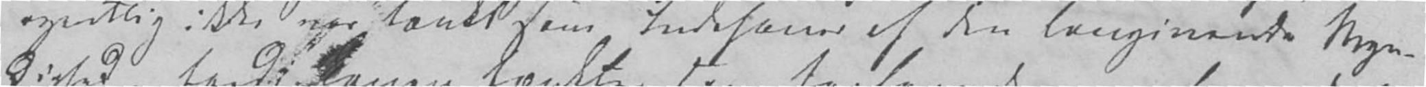egentlig ikke var tænkt som Indehaver af den lovgivende Myn-
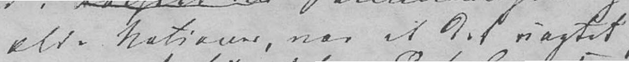ældre Nationer, var at det uagtet
